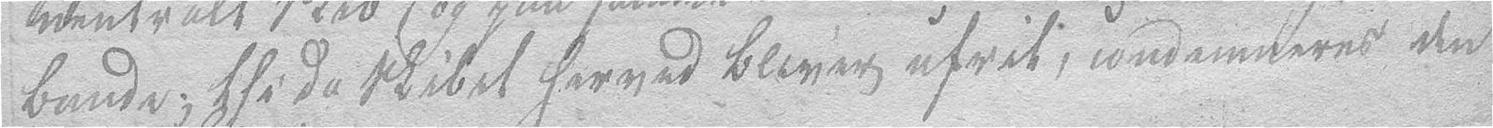bande; thi da Skibet herved bliver ufrit, condemneres den
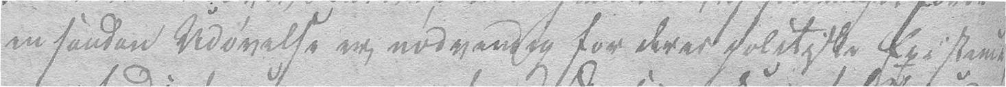en saadan Udøvelse er nødvendig for deres politiske Existence,
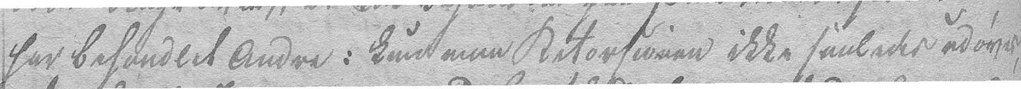har behandlet Andre: kun maa Retorsionen ikke saaledes udøves,
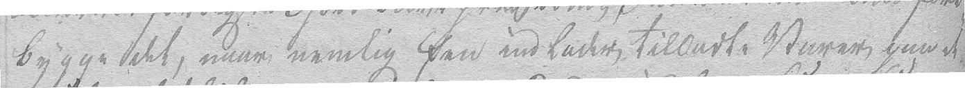bygge det, naar nemlig Een indlader tilladte Varer paa et
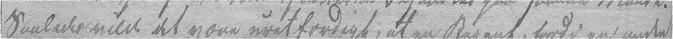Saaledes vilde det være uretfærdigt, at en Regent, fordi en anden
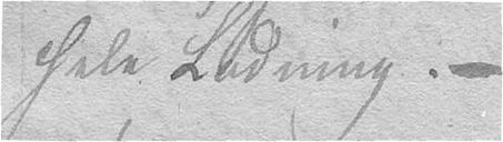hele Lodning. –
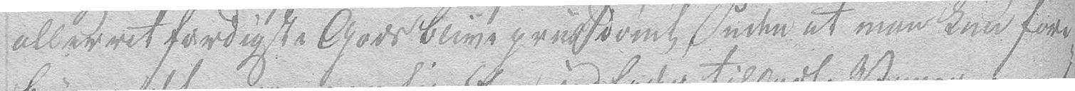aller retfærdigste Gods blive priisdømt uden at man kan fore-
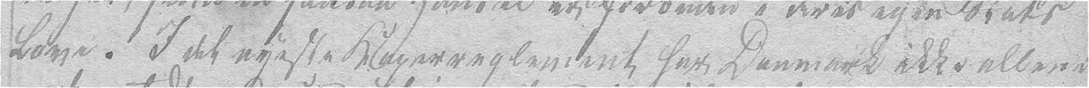Love. I det nyeste Magerreglement har Danmark ikke allene
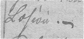Læsion. –
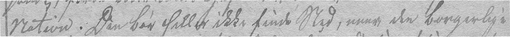Nation. Den bør heller ikke finde Sted, naar den borgerlige
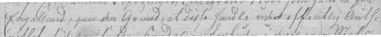Engelland, paa den Grund, at disse handle uden offentlig Autho-
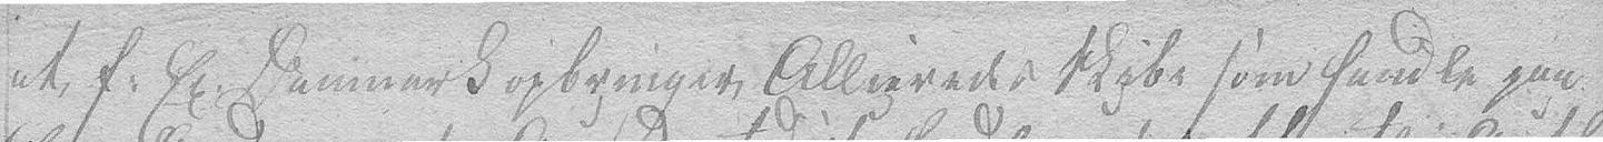at f: Ex: Danmark opbringer Allieredes Skibe som handle paa
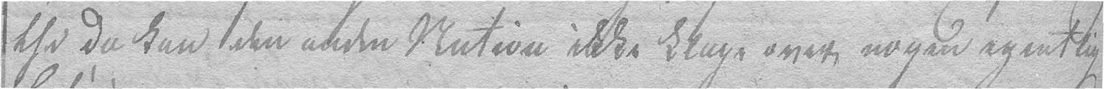thi da kan den anden Nation ikke Klage over nogen egentlig
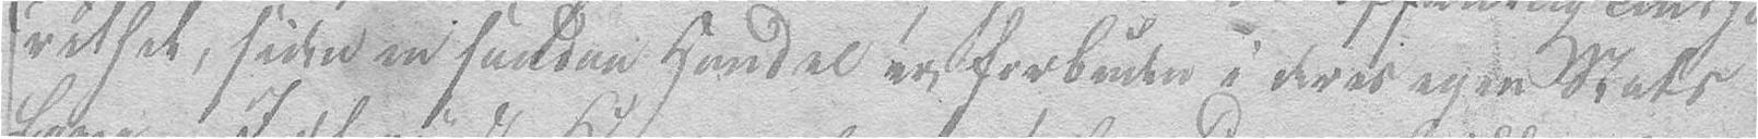ritet, siden en saadan Handel er forbuden i deres egen Stats
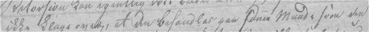ikke klage over, at den behandles paa samme Maade som den
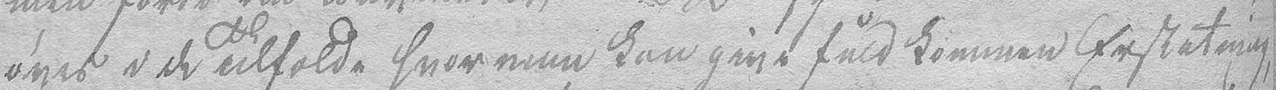øves i de Tilfælde hvor man kan give fuldkommen Erstatning,
